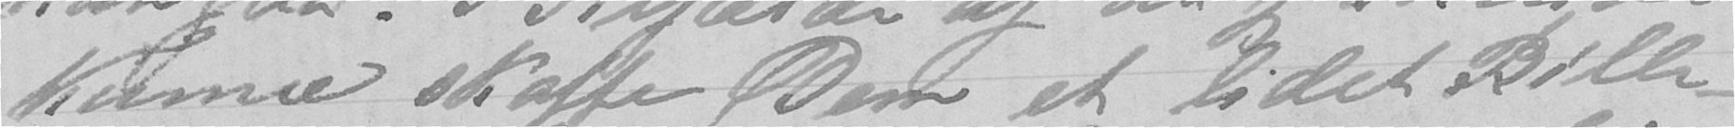kunne skaffe Dem et lidet Bille-
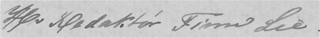Hr Redaktør Finn Lie.
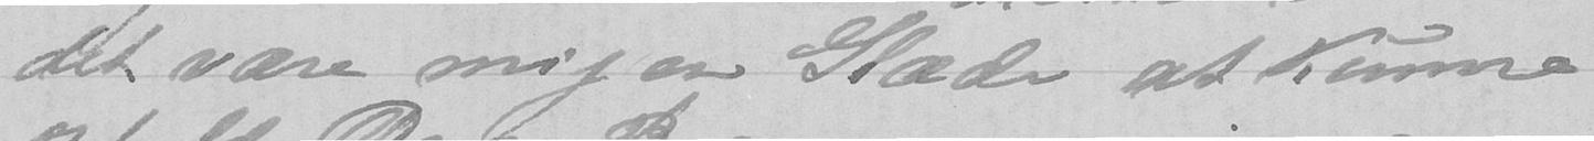det være mig en Glæde at kunne
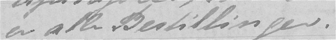er alle Bestillinger.
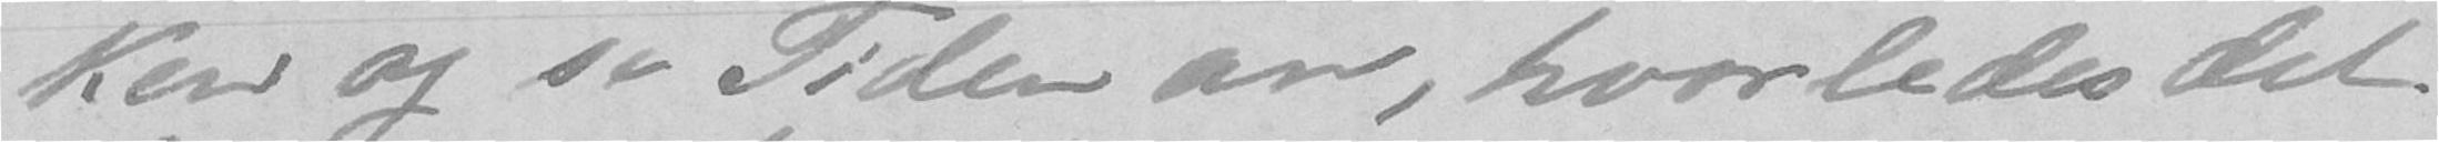ken og se Tiden an, hvorledes det
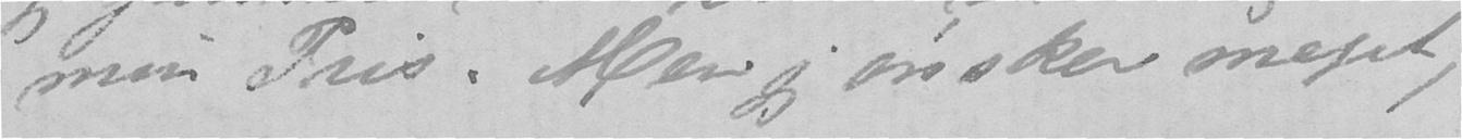min Pris. Men jeg ønsker meget,
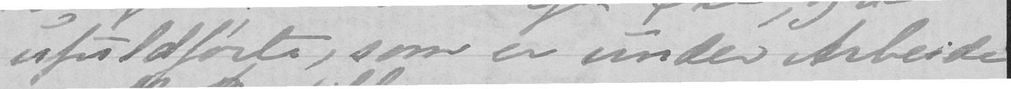ufuldførte, som er under Arbeide
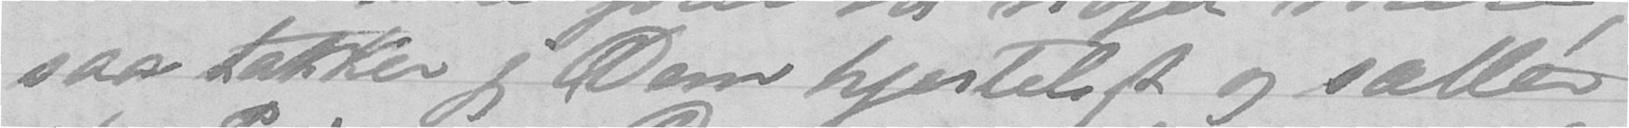saa takker jeg Dem hjerteligt og sætter
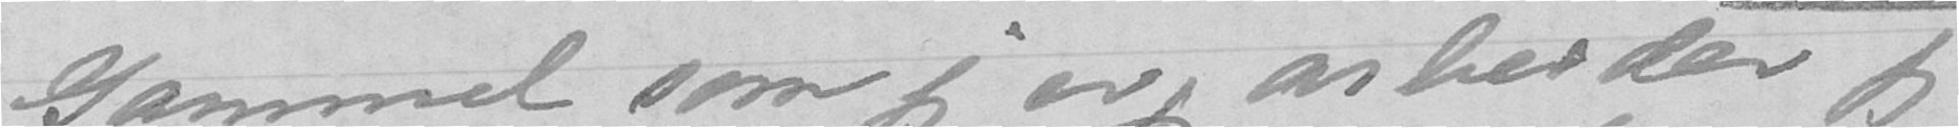Gammel som jeg er, arbeider jeg
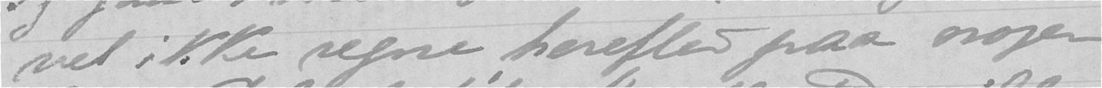vel ikke regne herefter paa nogen
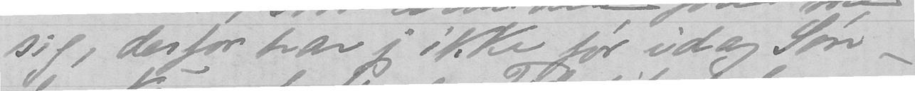sig, derfor har jeg ikke for idag Søn-
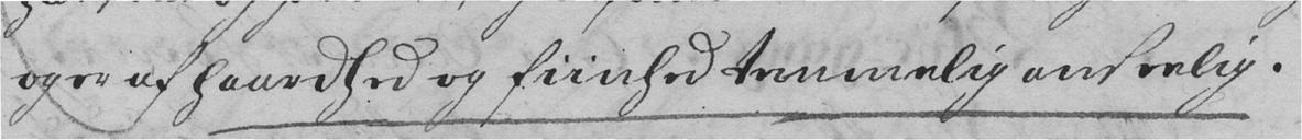og er af haardhed og fiinhed temmelig anseelig.
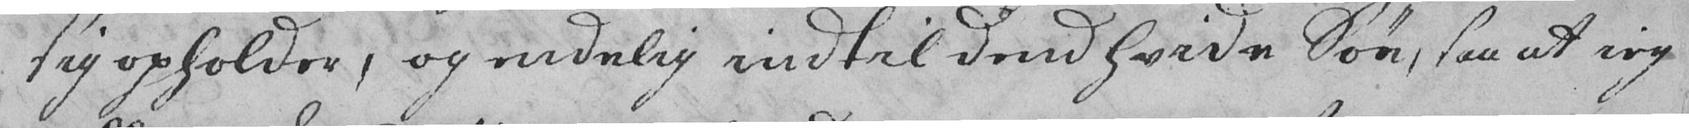sig opholder, og endelig indtil dend hvide Søe, saa at ieg
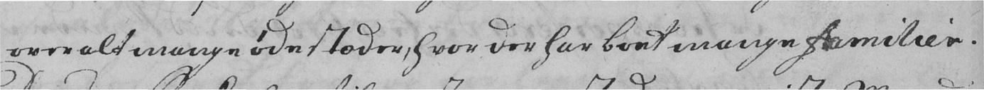over alt mange øde stæder, hvor der har boet mange familier.
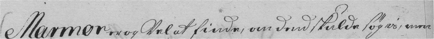Marmor er og Vel at finde, om dend skulde søgis, men
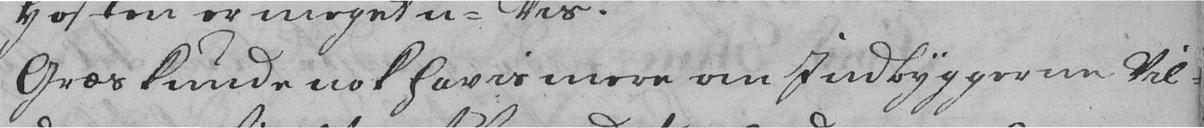Græs kunde nok havis mere om Indbyggerne Vil-
